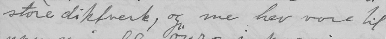store diktverk, og me hev vore til
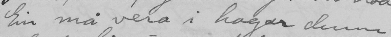Ein må vera i hagen denne
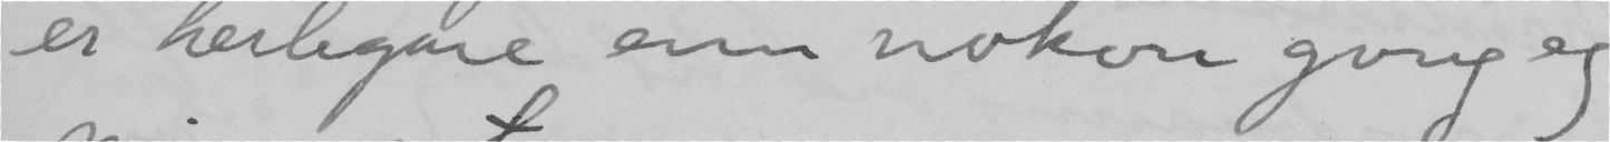er herlegare enn nokon gong og
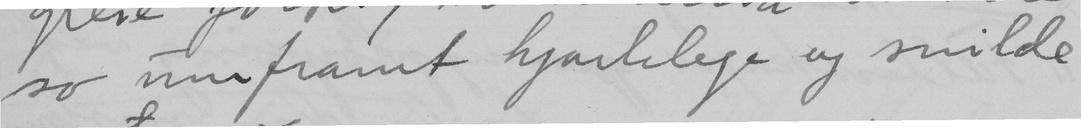so umframt hjartelege og snilde
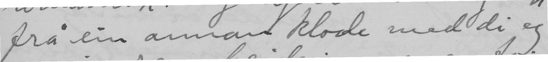frå ein annan klode med di eg
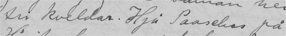tri kveldar. Hjå Paasches på
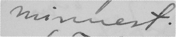minnest.
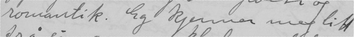romantik. Eg kjenner meg litt
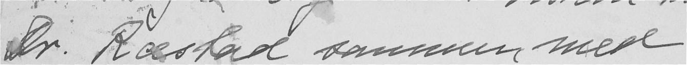Dr. Ræstad sammen med
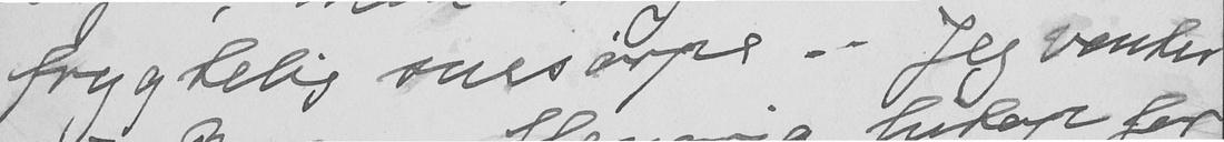frygtelig snesørpe. Jeg venter
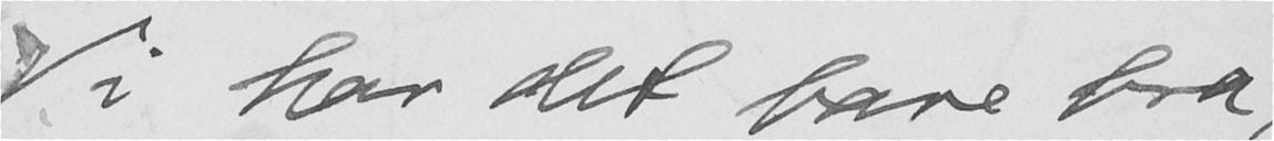Vi har det bare bra,
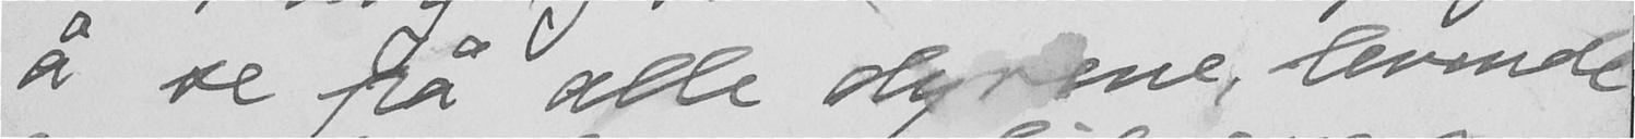å se på alle dyrene, levende
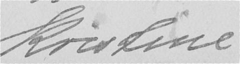Kristine
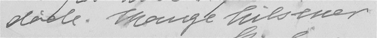og døde. Mange hilsener
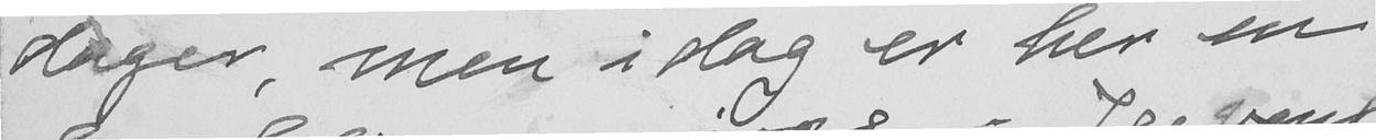dager, men i dag er her en
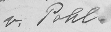v. Pohl.
